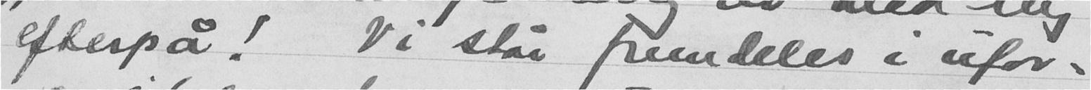efterpå. Vi står fremdeles i ufor-
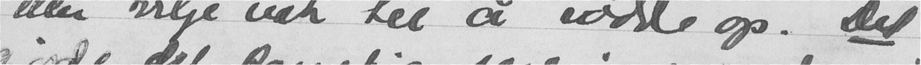eller vilje nok til å rødde op. Det
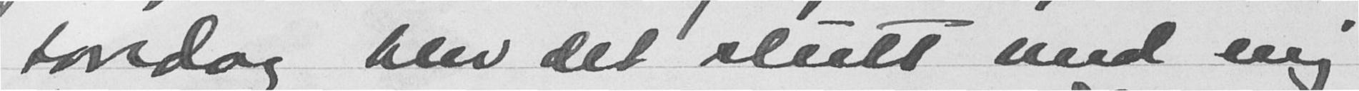torsdag blev det slutt med mig
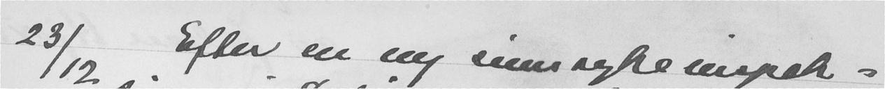23/12 Efter en ny sinnsykeinspek-
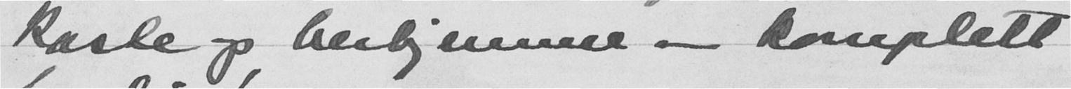kaste op herhjemme - komplett
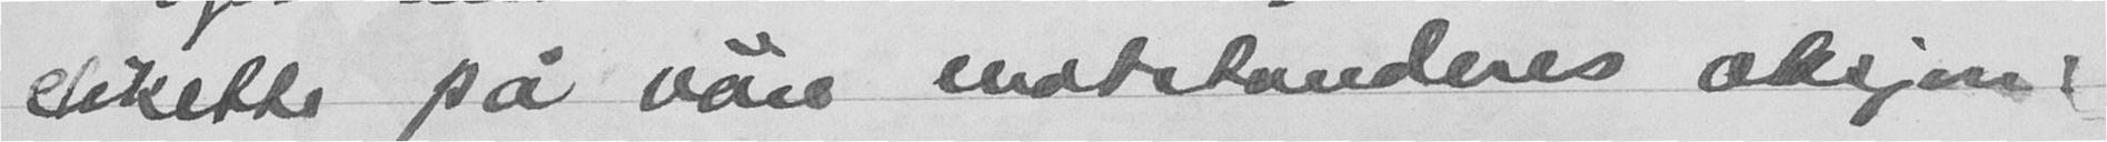elekette på våre motstanderes aksjon.
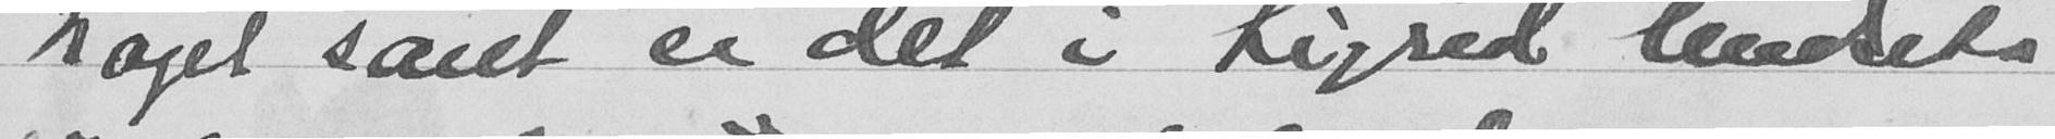Hagel sant er det i Sigrid Undsets
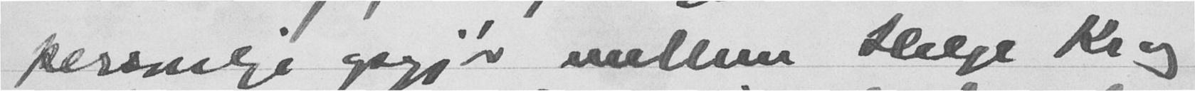personlige opgjør mellom Helge Krog
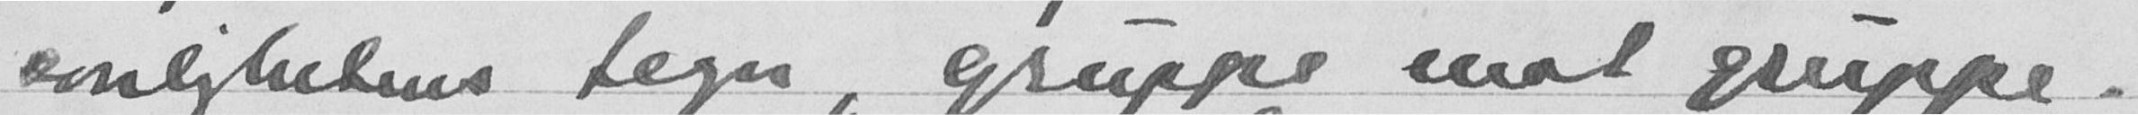sonelighetens tegn, gruppe mot gruppe.
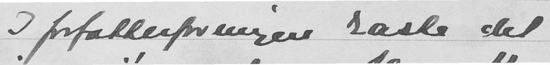I forfatterforeningen raste det
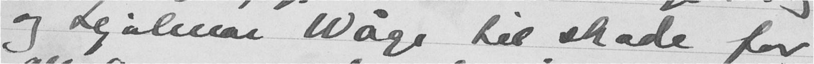og Hjalmar Wåge til skade for
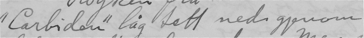"Carbiden" låg tett nedi gjenom
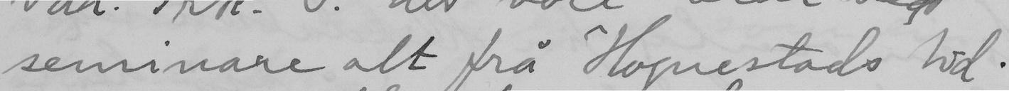seminarane alt frå Hognestads tid.
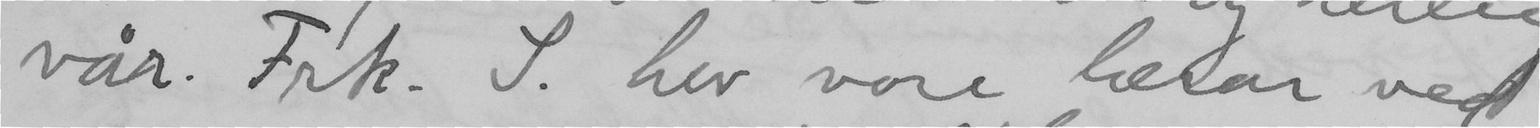vår. Frk. S. hev vore lærar ved
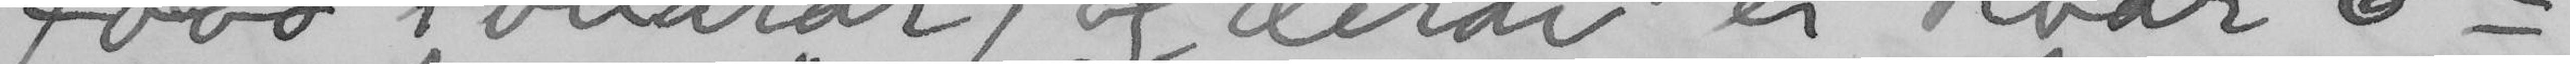7000 ibuarar, og dirar er kvar 6te
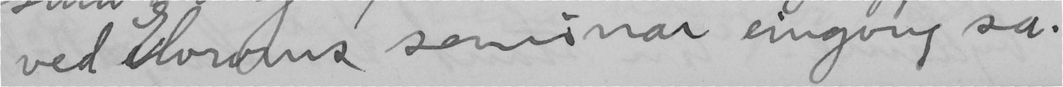ved loroms seminar eingong sa.
